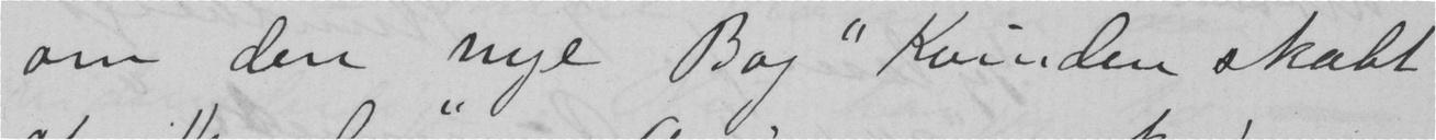om den nye Bog "Kvinden skabt
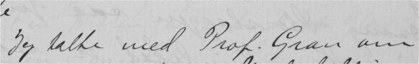Jeg talte med Prof. Gran om
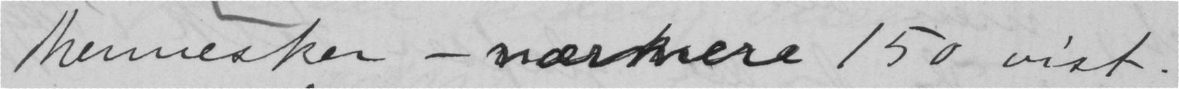Mennesker - nærmere 150 vist.
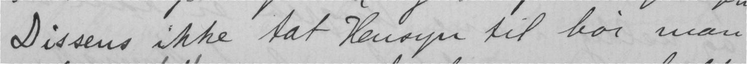Dissens ikke tat Hensyn til bør man
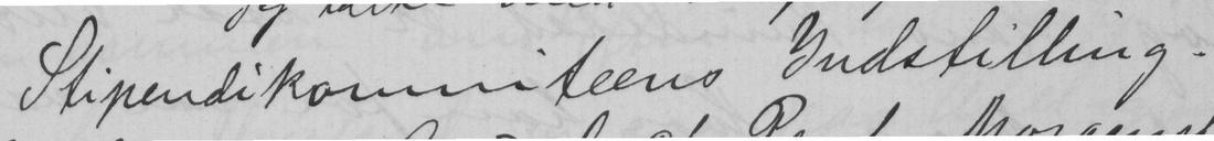Stipendikommiteens Indstilling -
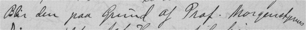Blir den paa Grund af Prof. Morgenstjernes
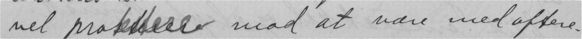vel protestere mod at være med oftere -
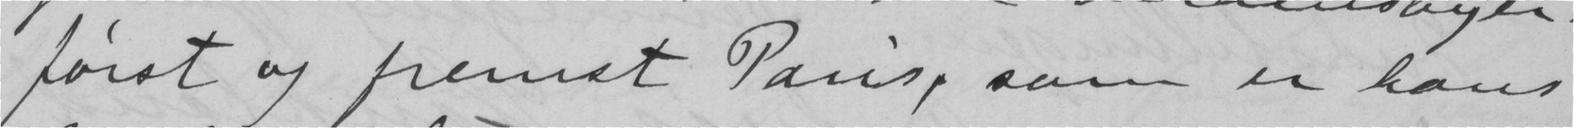først og fremst Paris, som er hans
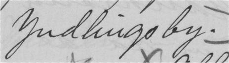yndlingsby.
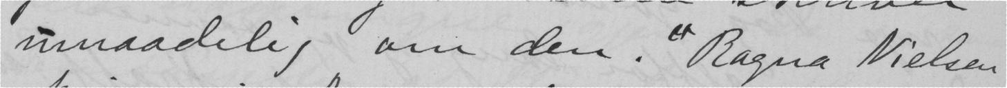umaadelig om den. "Ragna Nielsen
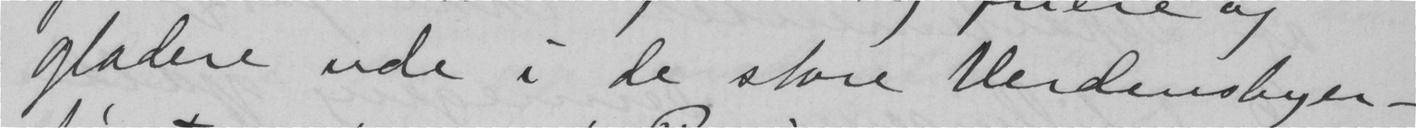gladere ude i de store verdensbyer -
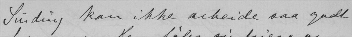Sinding kan ikke arbeide saa godt
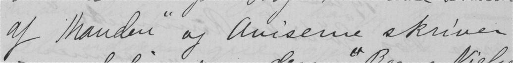af Manden" og Aviserne skriver
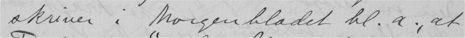skriver i Morgenbladet bl.a., at
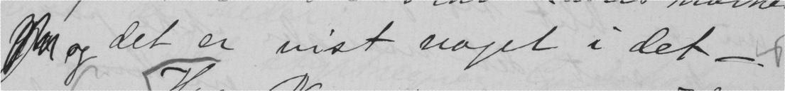og det er vist noget i det -.
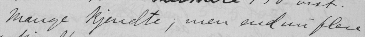mange kjendte; men endnu flere
